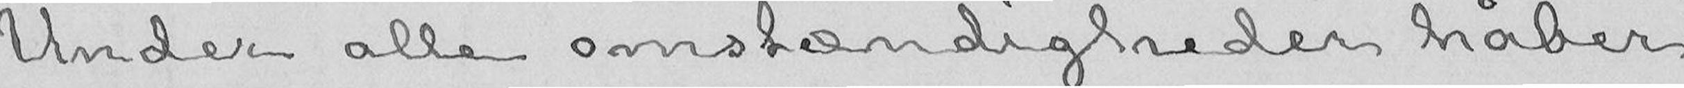Under alle omstændigheder håber
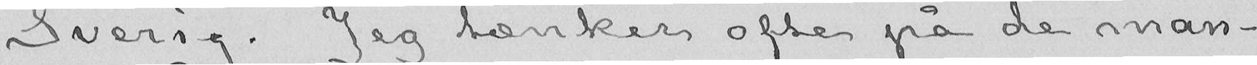Sverig. Jeg tænker ofte på de man-
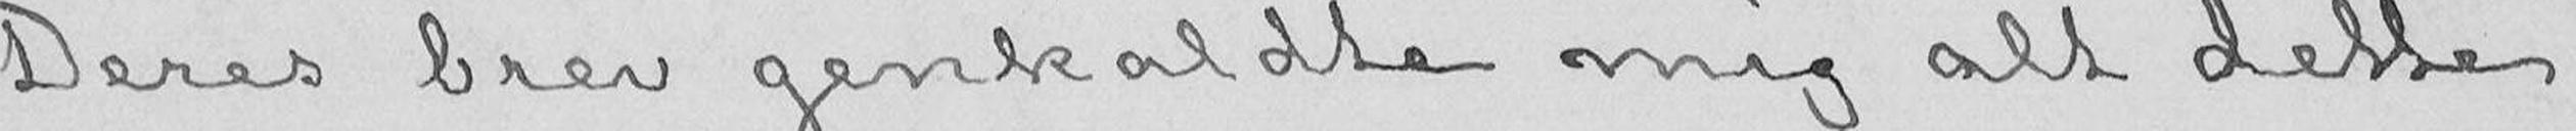Deres brev genkaldte mig alt dette
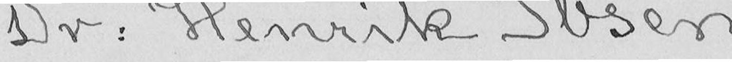Dr: Henrik Ibsen.
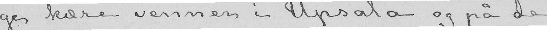ge kære venner i Upsala og på de
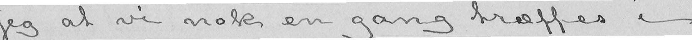jeg at vi nok en gang træffes i
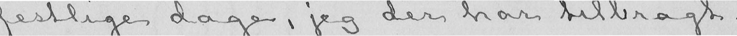festlige dage, jeg der har tilbragt.
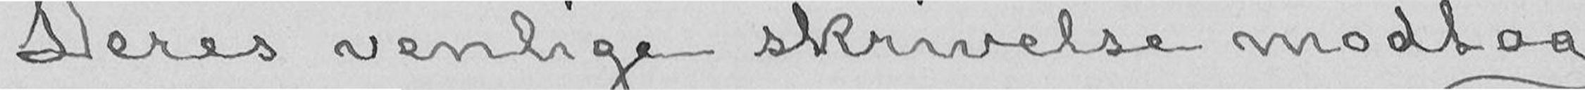Deres venlige skrivelse modtog
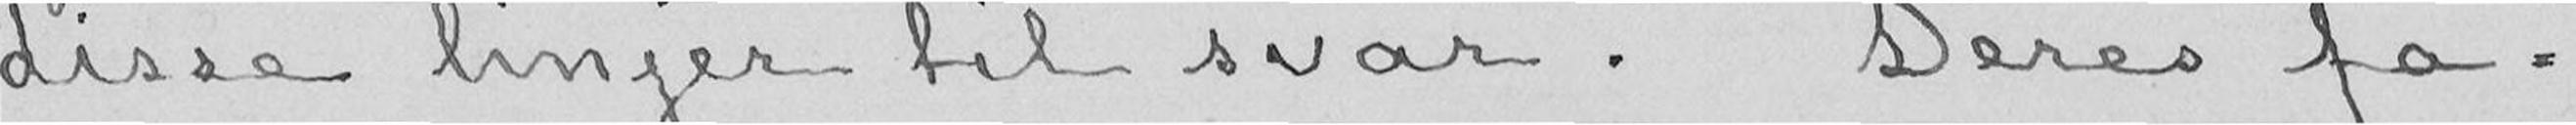disse linjer til svar. Deres fa-
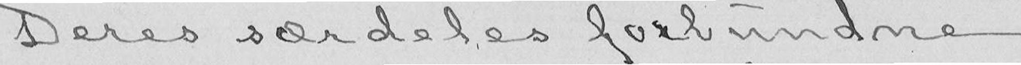Deres særdeles forbundne
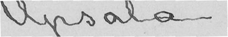Upsala.
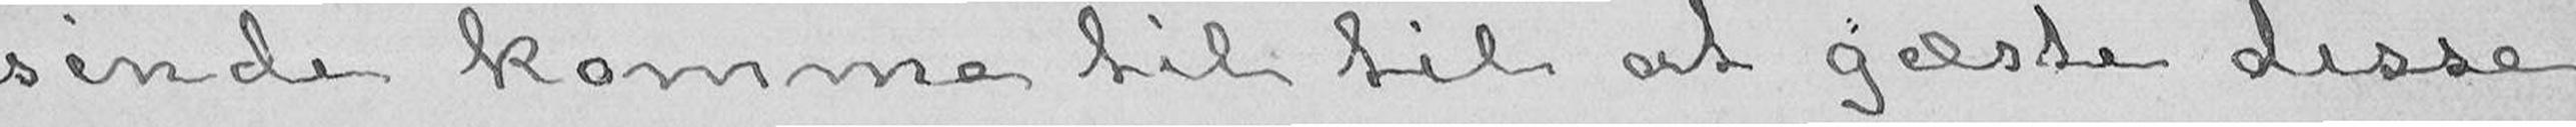sinde komme til til at gæste disse
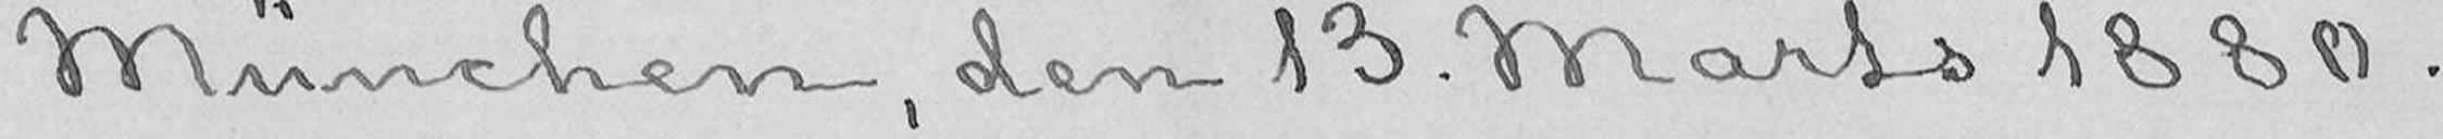München, den 13. Marts 1880.
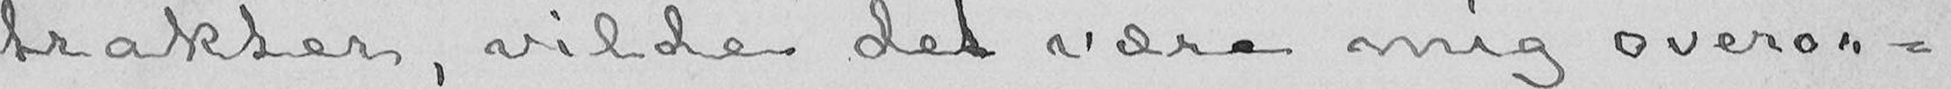trakter, vilde det være mig overor-
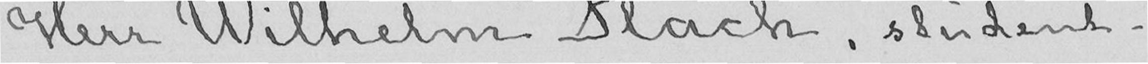Herr Wilhelm Flach, student.
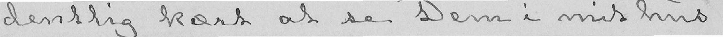dentlig kært at se Dem i mit hus.
